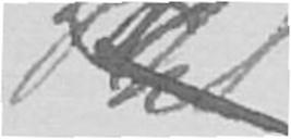til
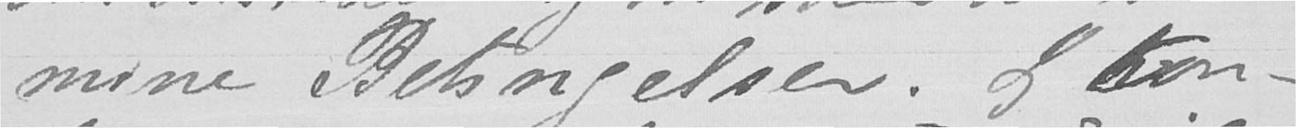mine Betingelser. Jeg kon-
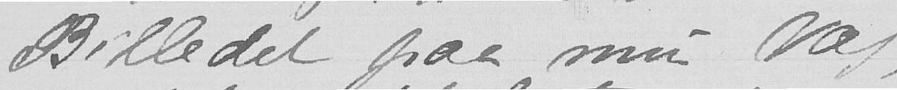Billedet paa min Væg,
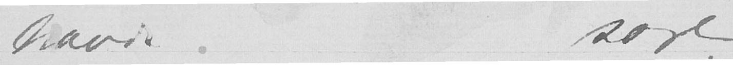havde sagt
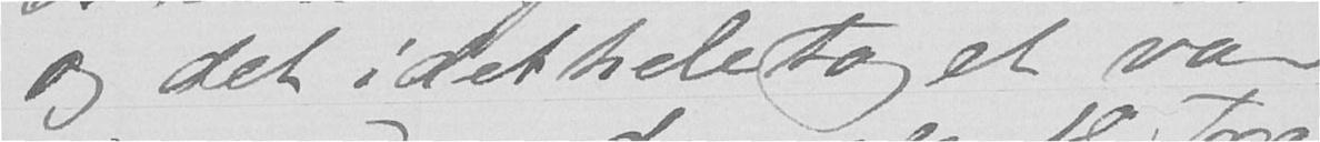og det idetheletaget var
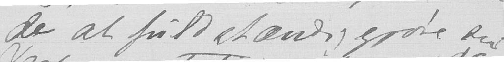de at fuldstændiggjøre den
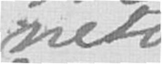netop
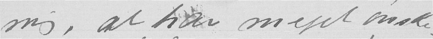mig, at han meget ønske-
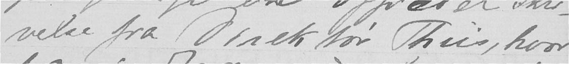velse fra Direktør Thiis, hvor
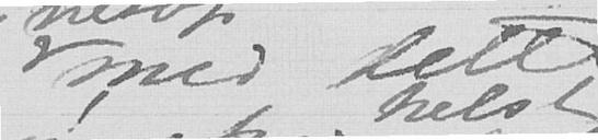nalgalleriet med dette
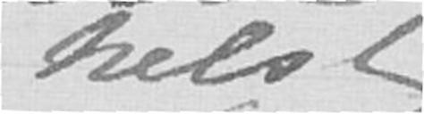helst
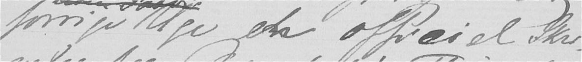forrige Uge en officiel Skri-
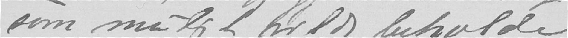som muligt vilde beholde
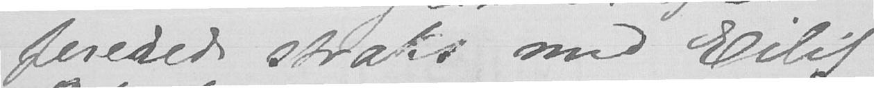fererede straks med Eilif
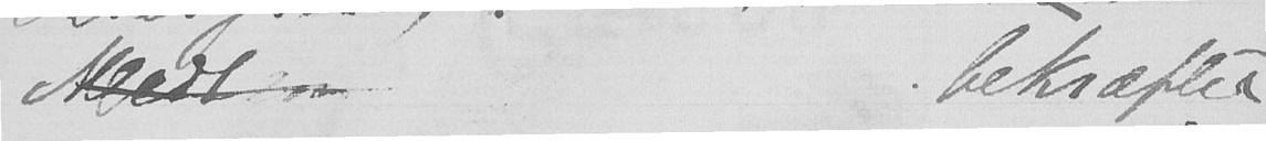Medlem bekræfter
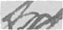for
